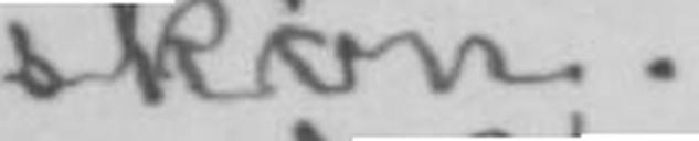skøn.
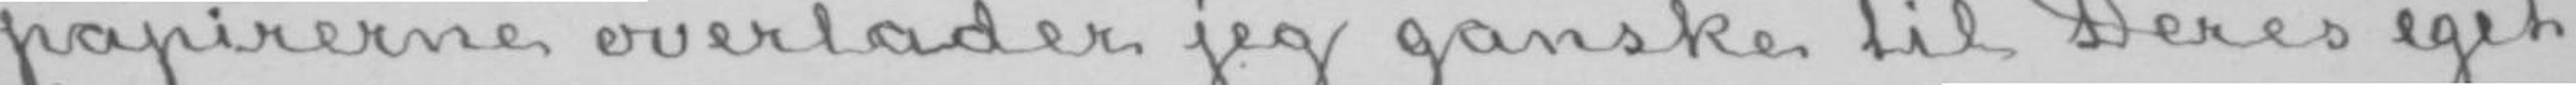papirerne overlader jeg ganske til Deres eget
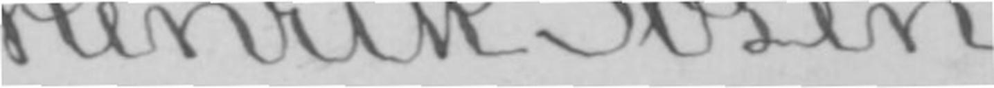Henrik Ibsen.
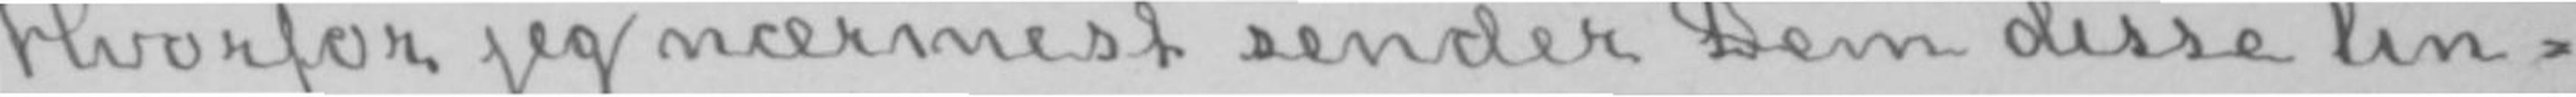Hvorfor jeg nærmest sender Dem disse lin-
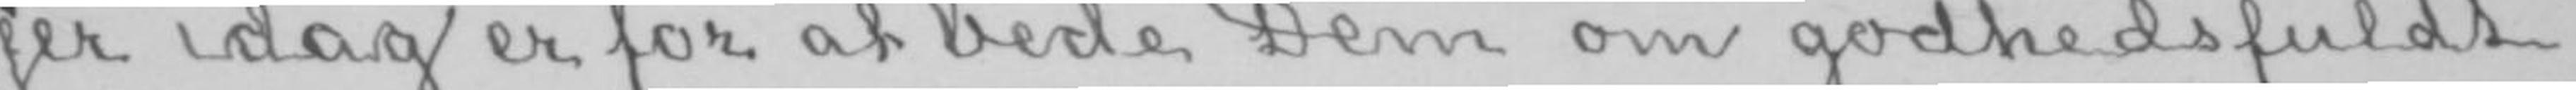jer idag er for at bede Dem om godhedsfuldt
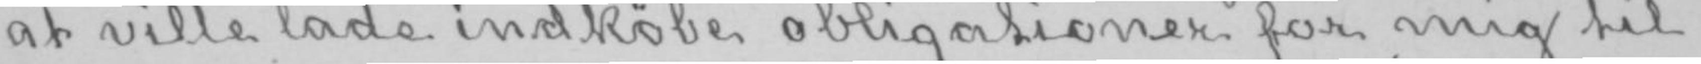at ville lade indkøbe obligationer for mig til
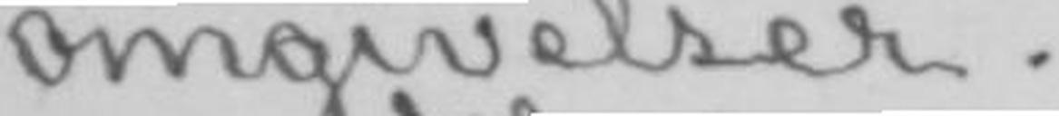omgivelser.
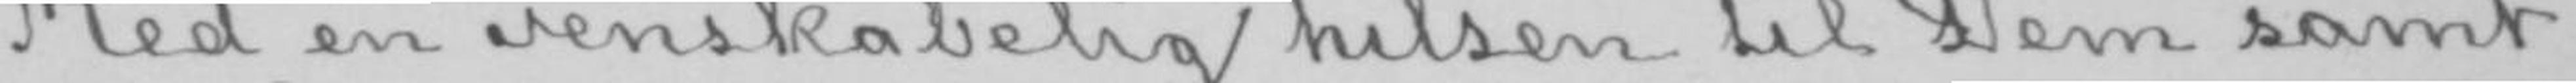Med en venskabelig hilsen til Dem samt
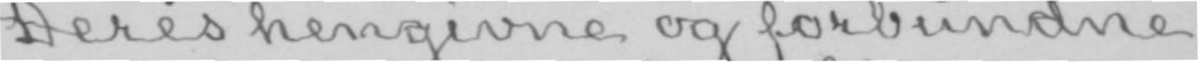Deres hengivne og forbundne
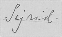Sigrid
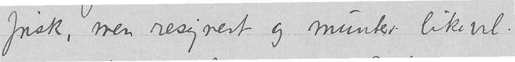frisk, men resignert og munter likevel.
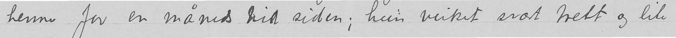henne for en måneds tid siden; hun virket svært trett og lite
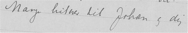Mange hilsener til Johan og dig
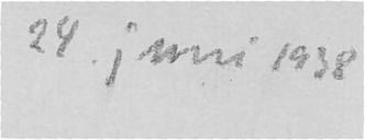24. juni 1938
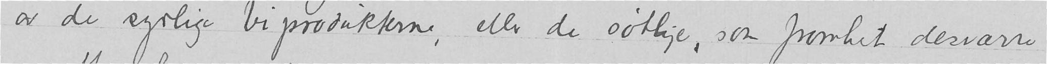av de syslige biprodukterne, eller de søtlige, som fromhet desværre
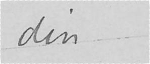din –
